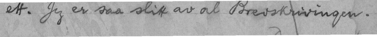ett. Jeg er saa slitt av al Brevskrivingen.
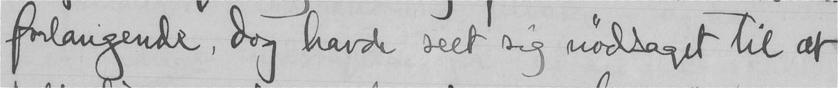forlangende, dog havde seet sig nødsaget til at
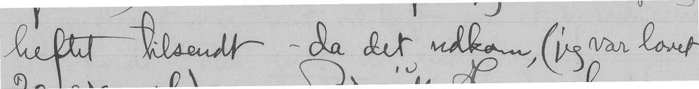heftet tilsendt - da det udkom, (jeg var lovet
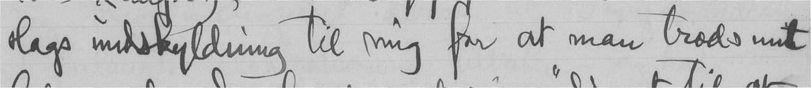slags unnskyldning til mig for at man trods mit
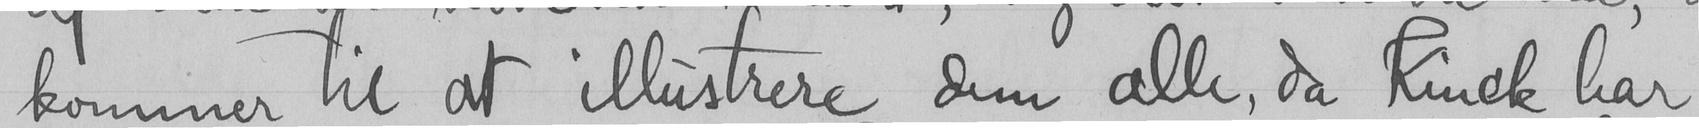kommer til at illustrere dem alle, da Kinck har
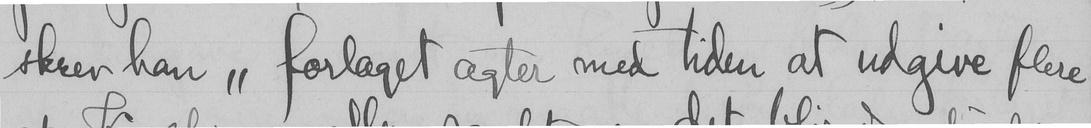skrev han "forlaget" agter med tiden at udgive flere
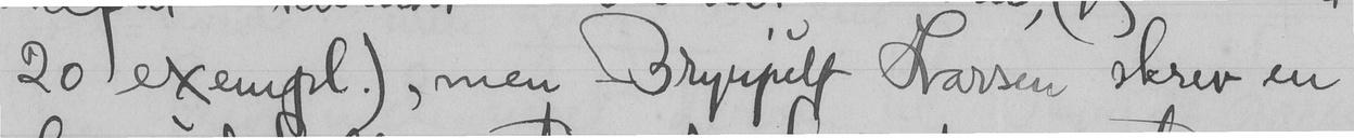20 exempl), men Brynjulf Larsen skrev en
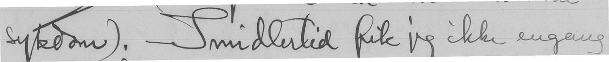sykdom). - Imidlertid fik jeg ikke engang,
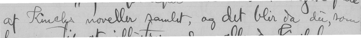af Kincks noveller samlet, - og det blir da du, som
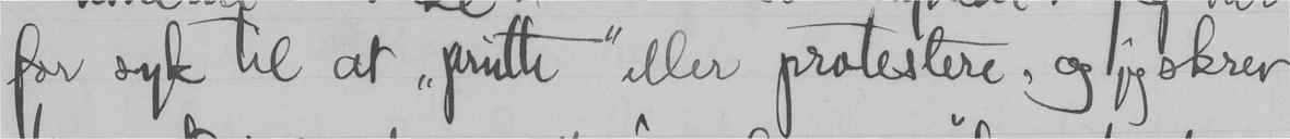for syk til at "prutte" eller protestere, og jeg skrev
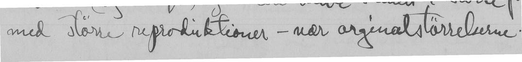med større reproduktioner - nær orginalstørrelserne.
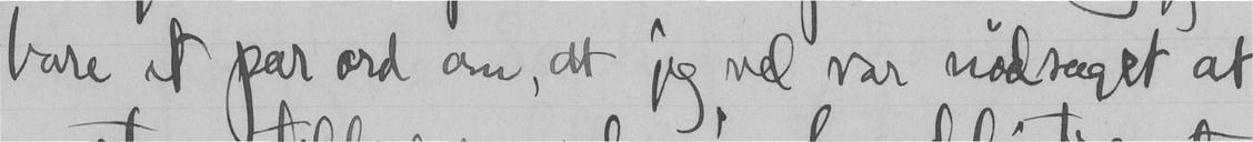bare at par ord om, at jeg vel var nødsaget at
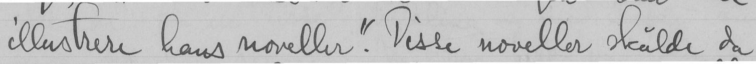illustrere hans noveller". Disse noveller skulde da
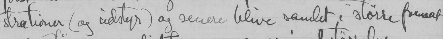strationer (og udstyr) og senere blive samlet i større format
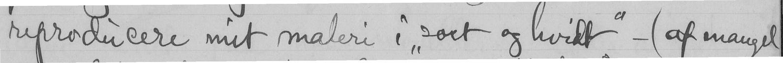reproducere mit maleri i "sort og hvidt" - (af mangel
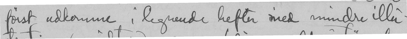først udkomme i legnende hefter med mindre illu-
